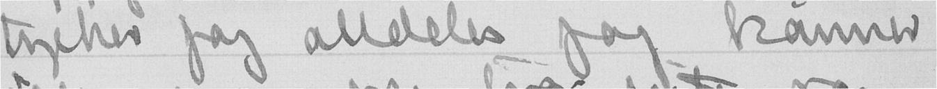tycker jag alleleles jag känner
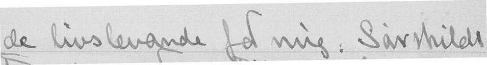de livslevande for mig. Särskilds
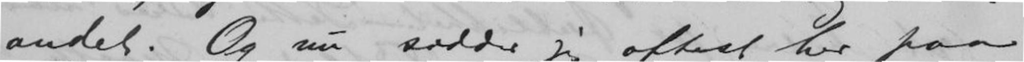andet. Og nu sidder jeg oftest her paa
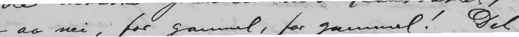- aa nei, for gammel, for gammel! Det
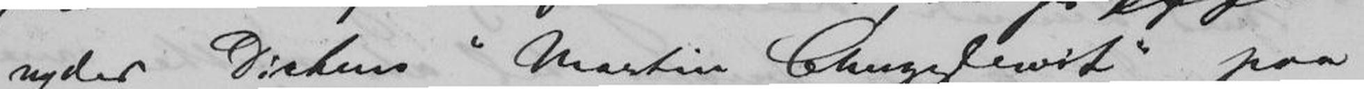nyder Diskens "Martin Chuzzlewit" paa
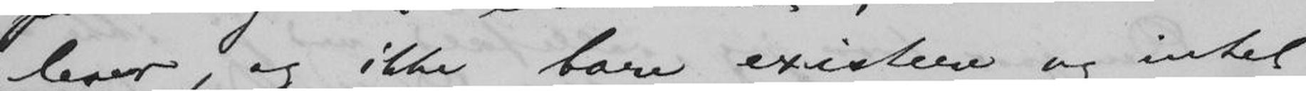lever, og ikke bare existere og intet
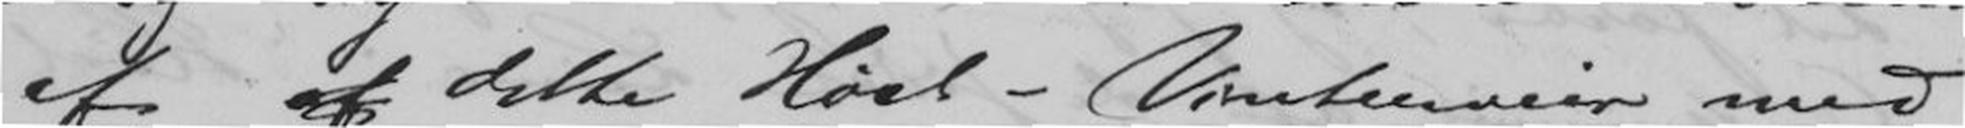af af dette Høst-Vinterveir med
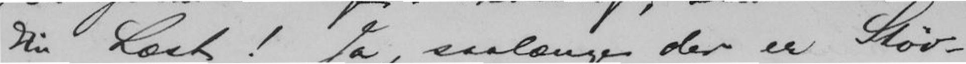Din Læst! Ja, saalænge der er Støv-
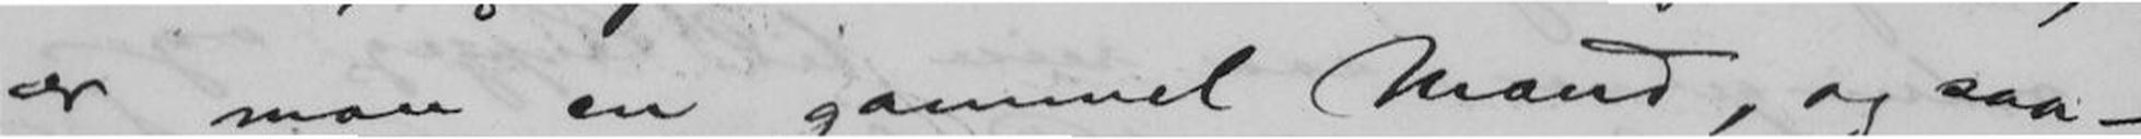er man en gammel Mand, og saa -.
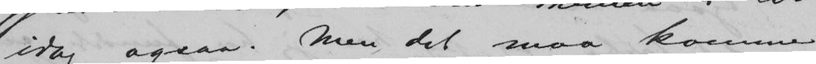idag ogsaa. Men det maa komme
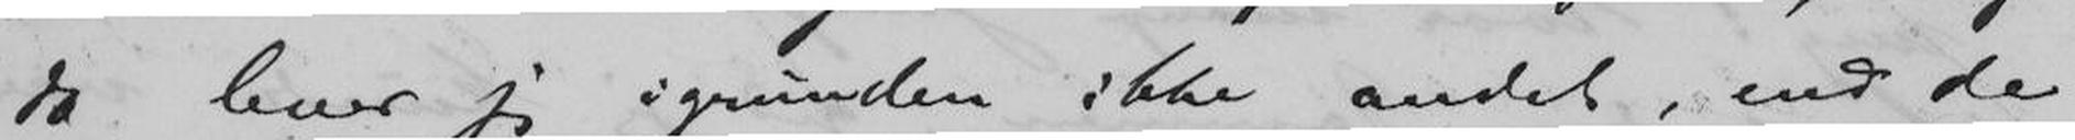da lever jeg igrunden ikke andet, end de
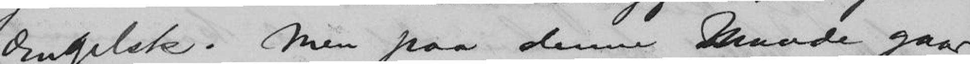Engelsk. Men paa denne Maade gaar
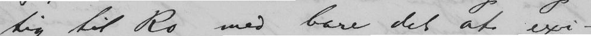tig til Ro med bare det at exi-
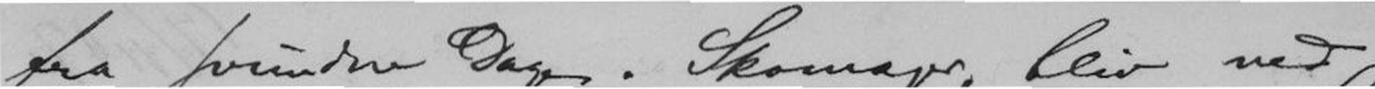fra svundne Dage. Skomager, bliv ved
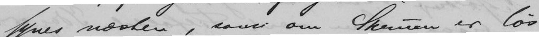synes næsten, som om Skruen er løs
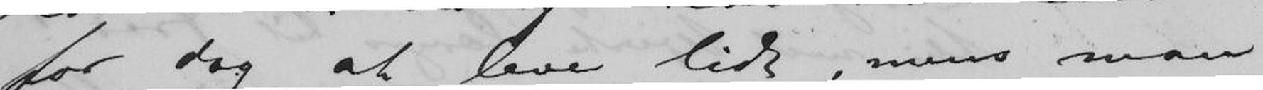for dog at leve lidt, mens man
